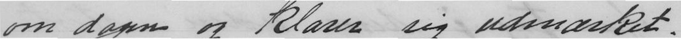om dagen og klarer sig udmærket.
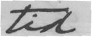tid
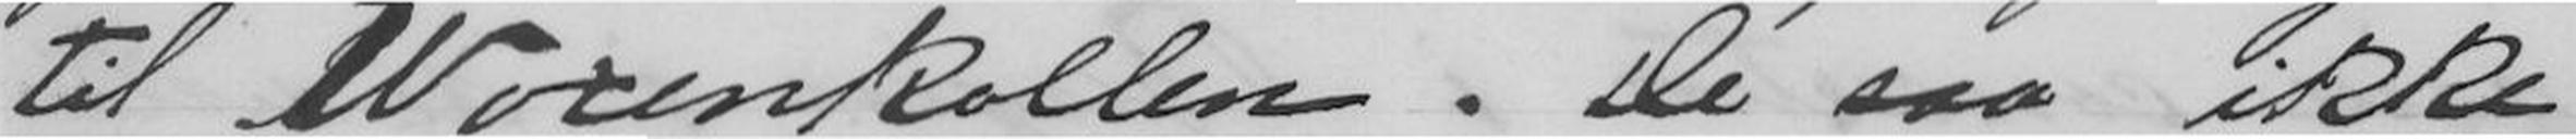til Woxenkollen. De saa ikke
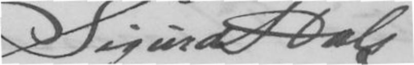Sigurd Hals
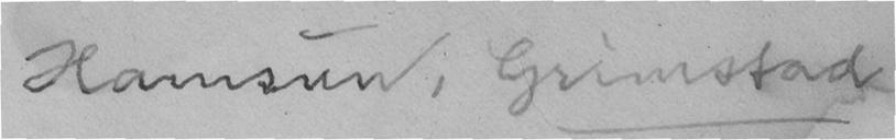Hamsun, Grimstad
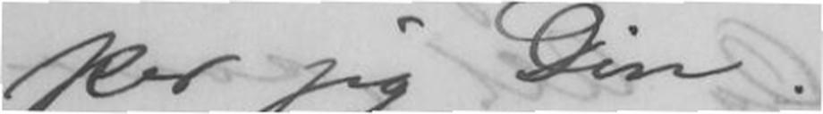per jeg Din.
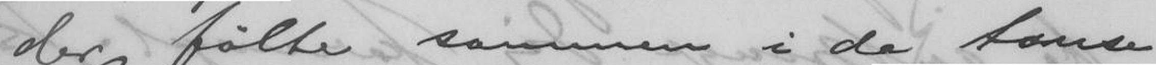der følte sammen i de tause
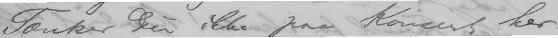Tænker Du ikke paa Konsert her
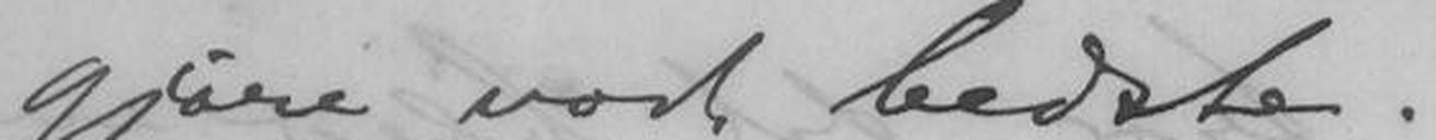gjøre vort bedste.
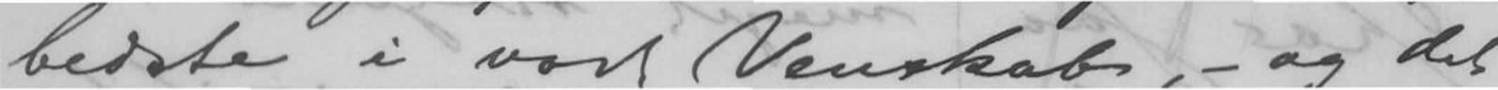bedste i vort Venskab, - og det
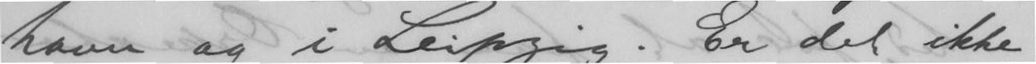havn og Leipzig. Er det ikke
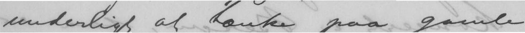underligt at tænke paa gamle
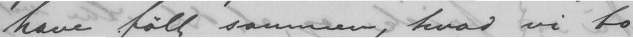have følt sammen, hvad vi to
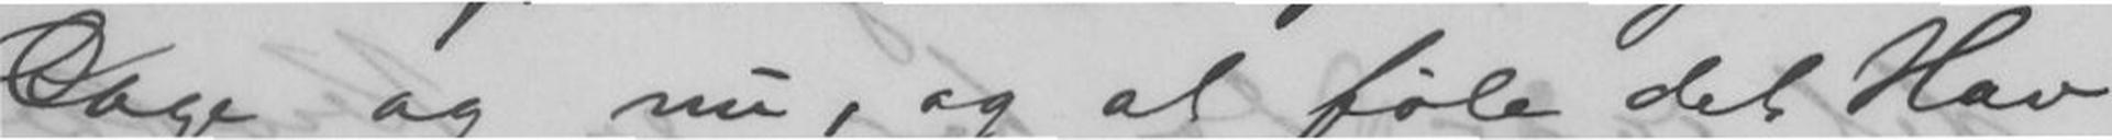Dage og nu, og at føle det Hav
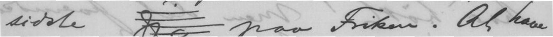sidste paa Friken. At have
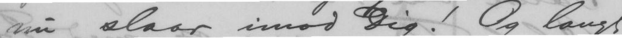nu slaar imod Dig! Og langt
